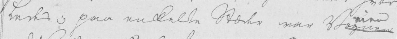ledes; paa enkelte Stæder var Vognen
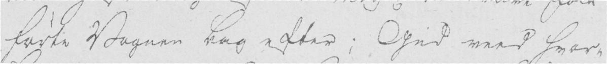førte Vognen bag efter; Gud veed hvor-
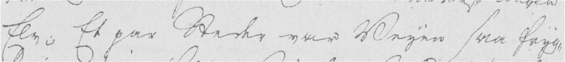Elv; Et par Steder var Veyen saa fryg-
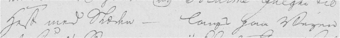Hest med Slæden - langs paa Veyen
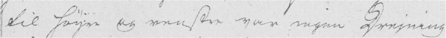til høyre og venstre var ingen Drejning
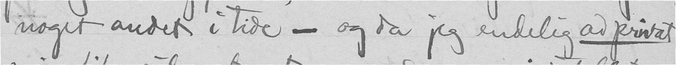noget andet i tide - og da jeg endelig av privat
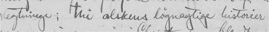slegtninge; thi alskens løgnagtige historier
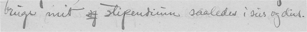bruge mit  stipendium saaleder i sus og dus.
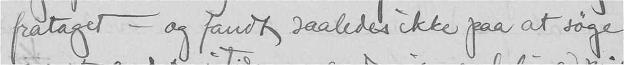frataget - og fandt saaledes ikke paa at søge
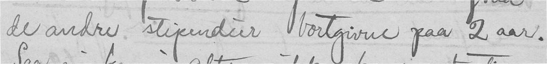de andre stipendier bortgivne paa 2 aar.
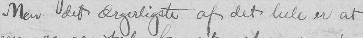Men det ærgerligste af det hele er at
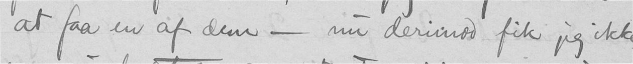at faa en af dem - nu derimod fik jeg ikke
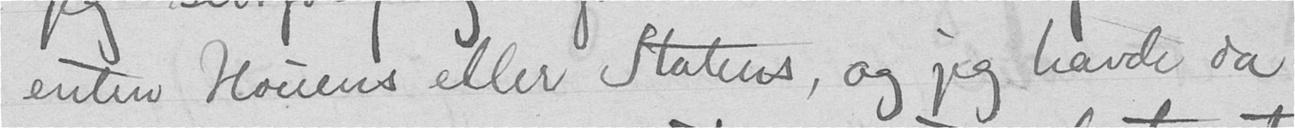enten Houens eller Statens, og jeg havde da
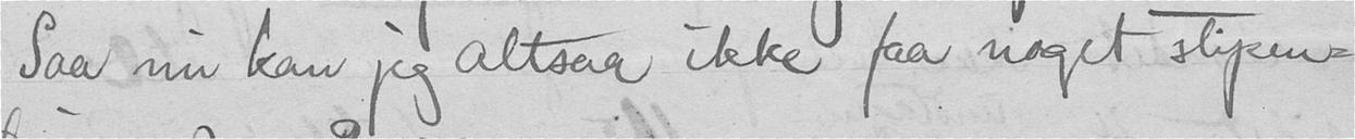Saa nu kan jeg altsaa ikke faa noget stipen-
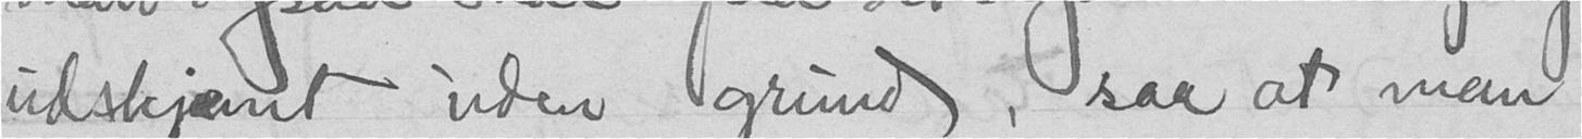udskjæmt uden grund, - saa at man
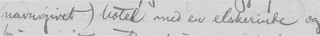(navngivet) hotel med en elskerinde og
